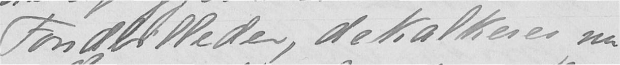Fondbilleder, dakalkeres nu
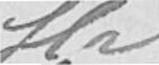Hr
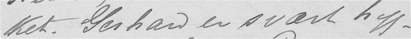ket. Gerhard er svært hyg-
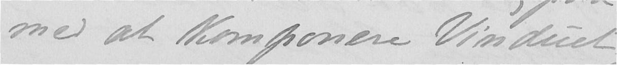med at komponere Vinduet,
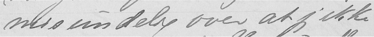misundelig over at jeg ikke
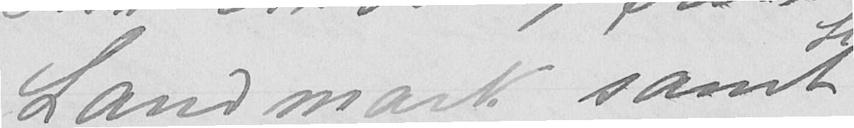Landmark samt
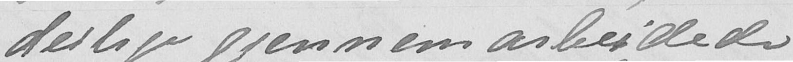deilige gjennemarbeidede
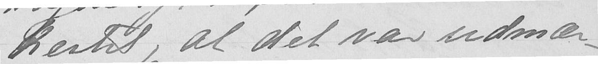Bertil, at det var udmær-
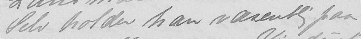Selv holder han væsentlig paa
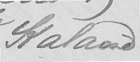Kaland.
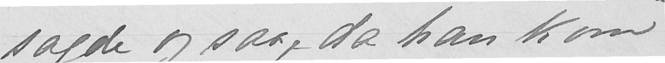sagde ogsaa, da han kom
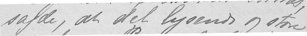sagde, at det lysende og store
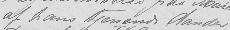af hans tjenende Aander,
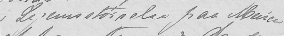i Legemsstørrelse paa Muren
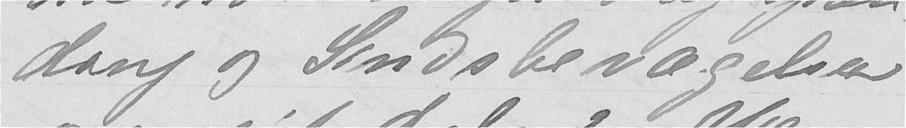ding og Sindsbevægelser
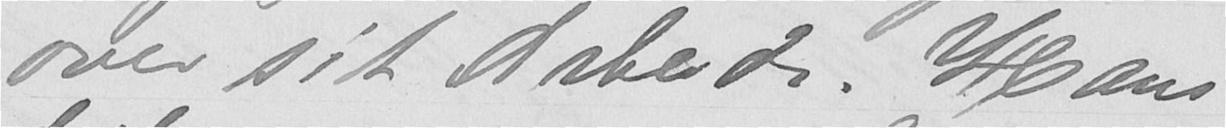over sit Arbeide. Hans
